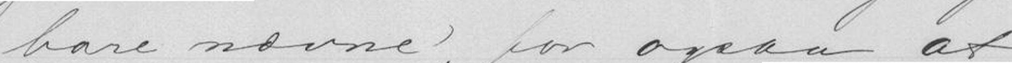bare nævne; for ogsaa at
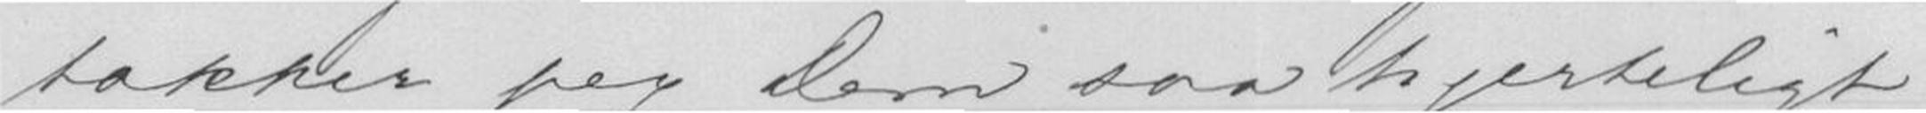takker jeg Dem saa hjerteligt
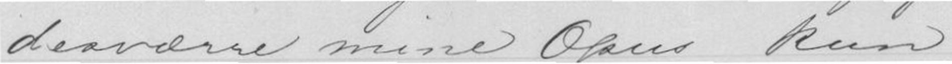desværre mine Opus kun
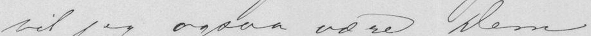vil jeg ogsaa være Dem
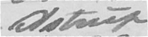Astrup
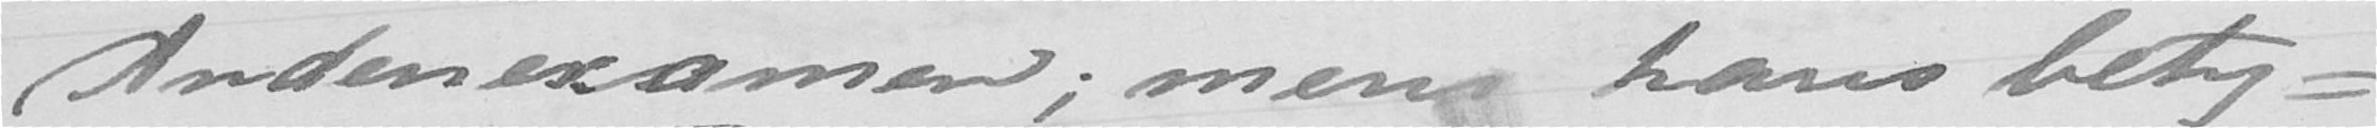Andenexamen; men hans bety-
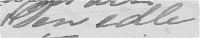Den edle
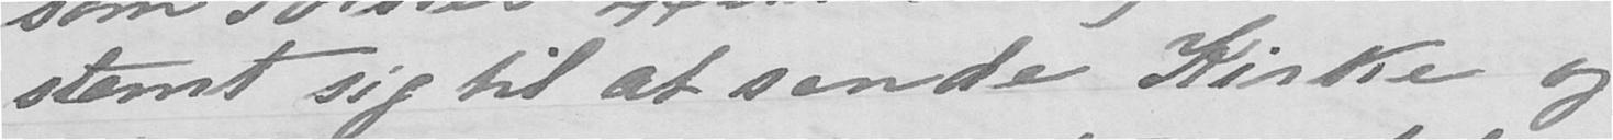stemt sig til at sende Kirke og
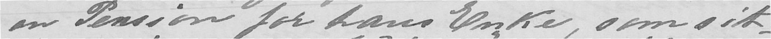en Pension for hans Enke, som sit-
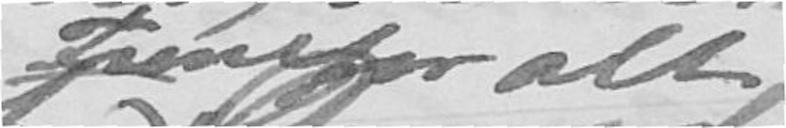Fremfor alt
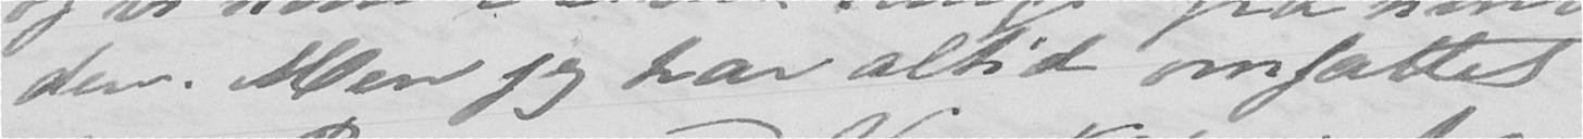den. Men jeg har altid omfattet
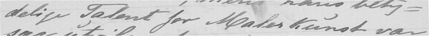delige Talent for Malerkunst var
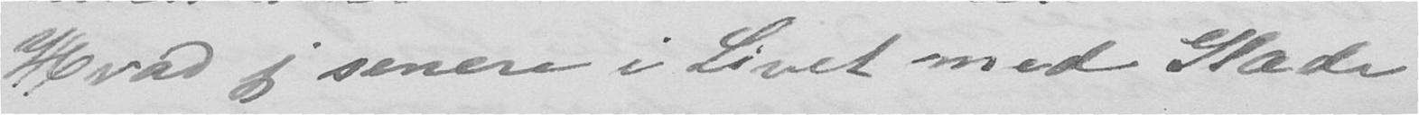Hvad jeg senere i Livet med Glæde
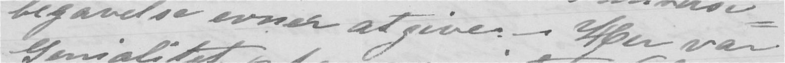begavelse evner at give. - Her var
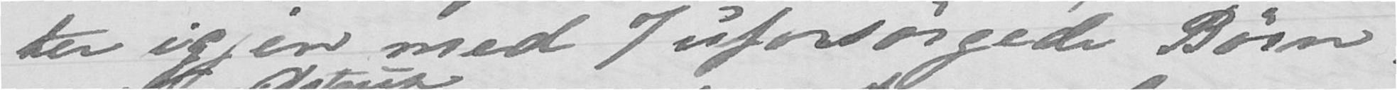ter igjen med 7 uforsørgede Børn.
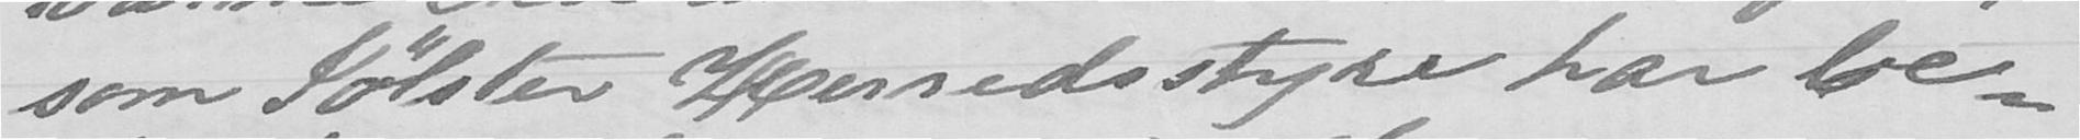som Jølster Herredsstyre har be-
